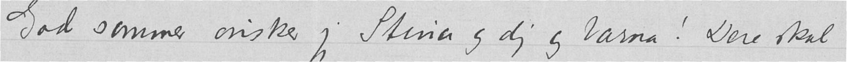God sommer ønsker jeg Stina og dig og barna! Dere skal
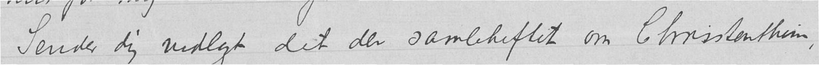Sender dig vedlagt det der samleheftet om Christenthum,
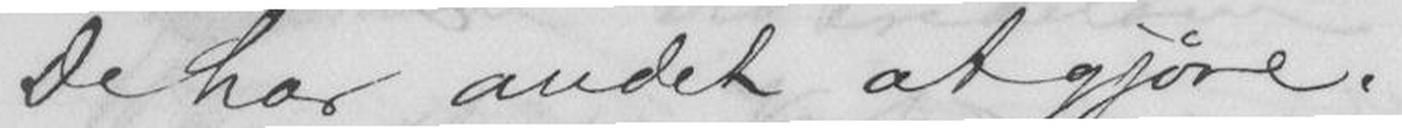De har andet at gjøre. -
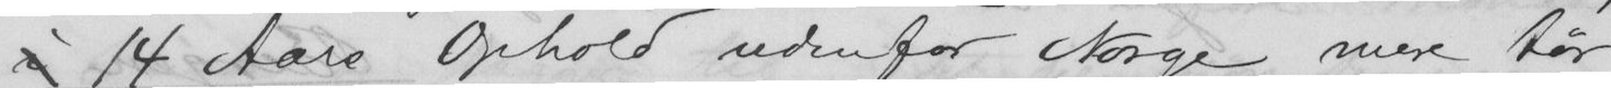i 14 Aars Ophold udenfor Norge mere tør
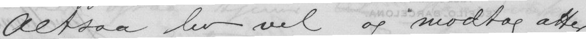Altsaa lev vel og modtag atter
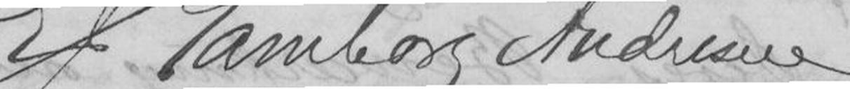E J Gamborg Andresen
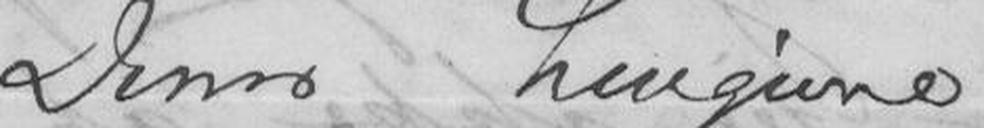Deres hengivne
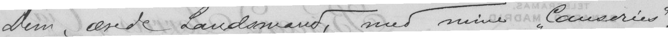Dem, ærede Landsmand, med mine "Causeries";
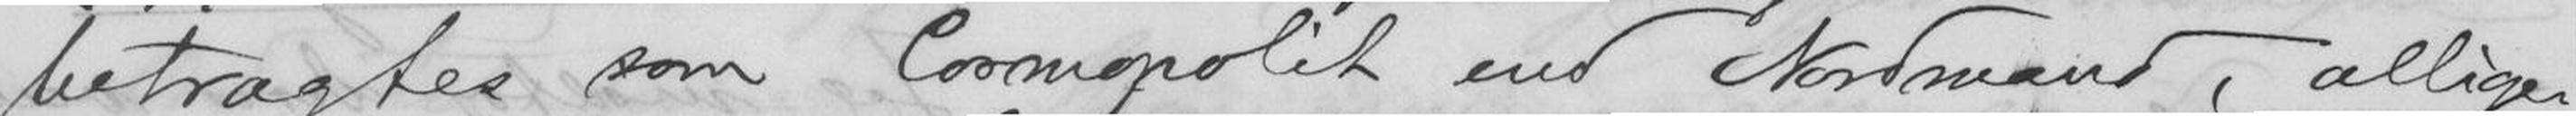betragtes som Cosmopolit end Nordmand, alligevel
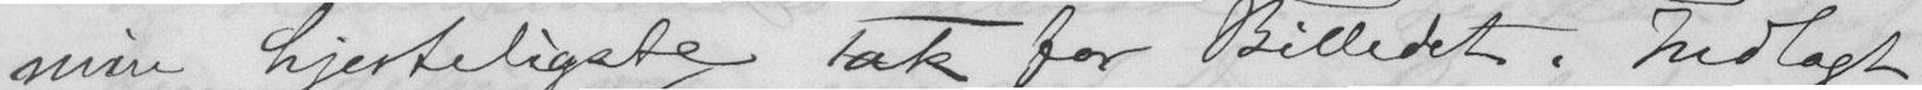min hjerteligste Tak for Billedet. Indlagt
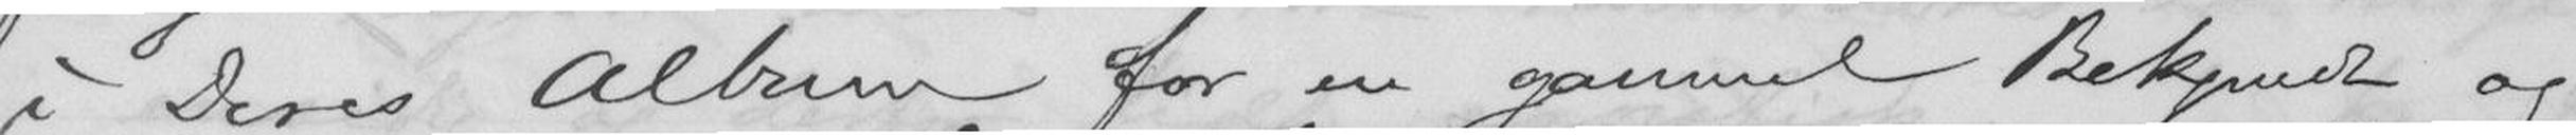i Deres Album for en gammel Bekjendt og
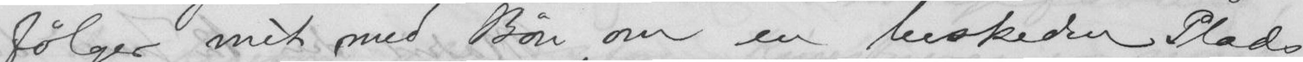følger mit med Bøn om en beskeden Plads
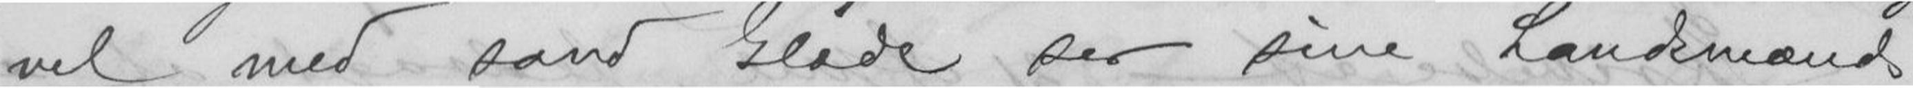med sand Glæde ser sine Landsmænds
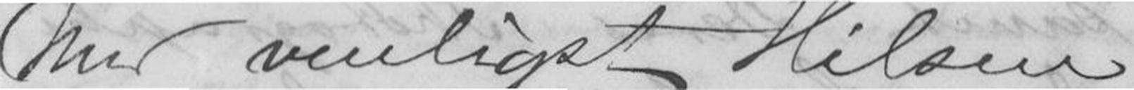Med venligst Hilsen
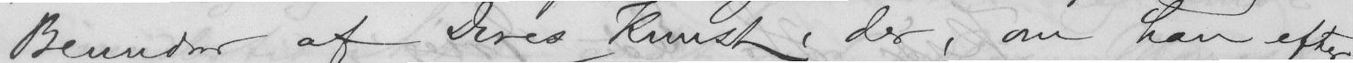Beundrer af Deres Kunst, der, om han efter
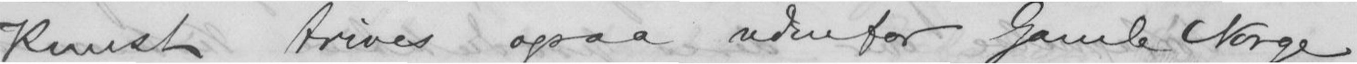Kunst trives ogsaa udenfor Gamle Norge
